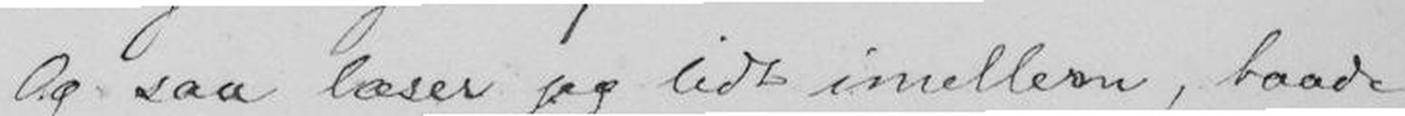Og saa læser jeg lidt imellem, baade
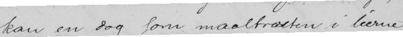kan en dog som maaltrosten i lierne
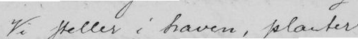Vi steller i haven, planter
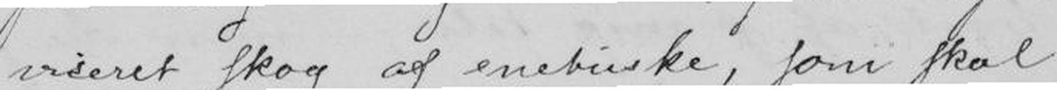viseret skog af enebuske, som skal
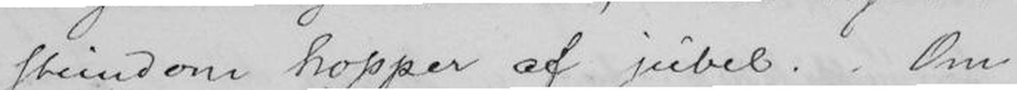stundom hopper af jubel. Om
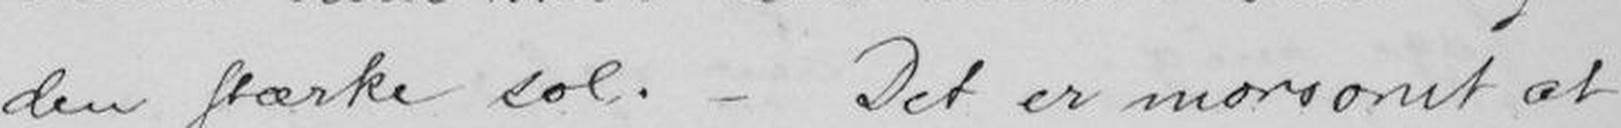den stærke sol. - Det er morsomt at
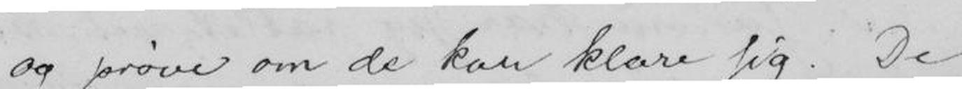og prøve om de kan klare sig. De
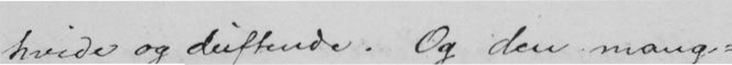hvide og duftende. Og den mang-
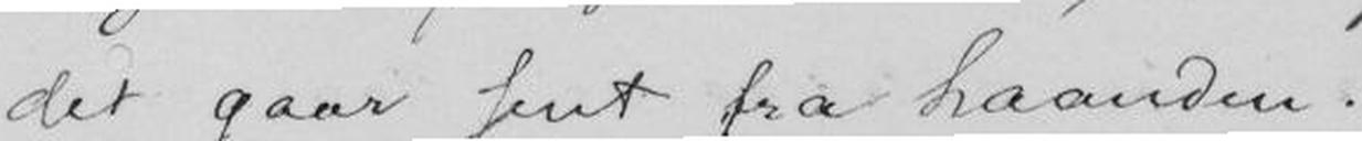det gaar fint fra haanden,
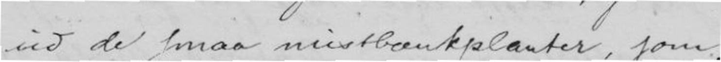ud de smaa mistbankplanter, som,
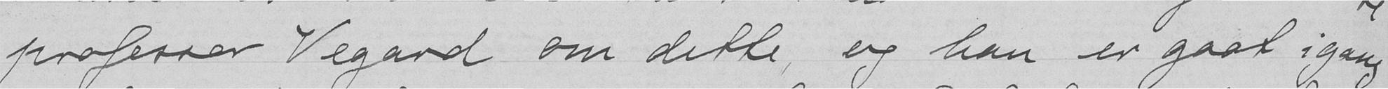professor Vegard om dette, og han er gaat igang
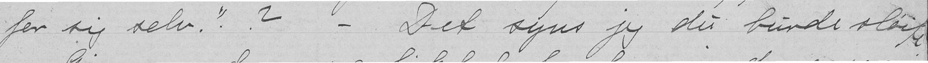fer sig selv." ? - Det syns jeg du burde sløife
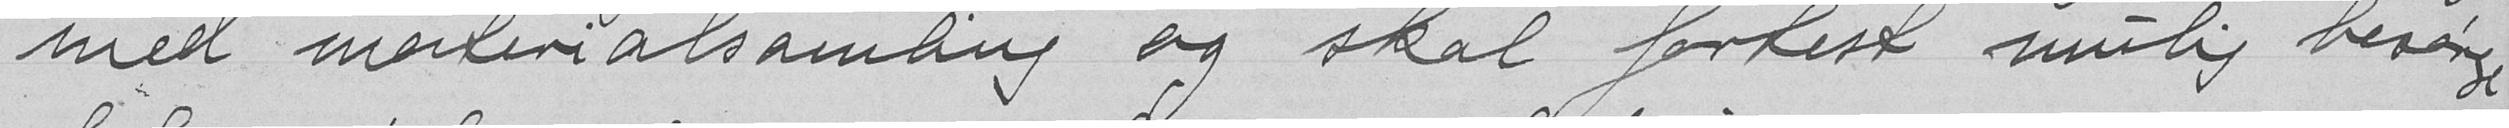med materialsamling og skal fortest mulig besøge
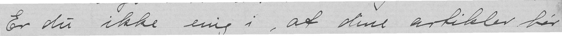Er du ikke enig i, at dine artilkler bør
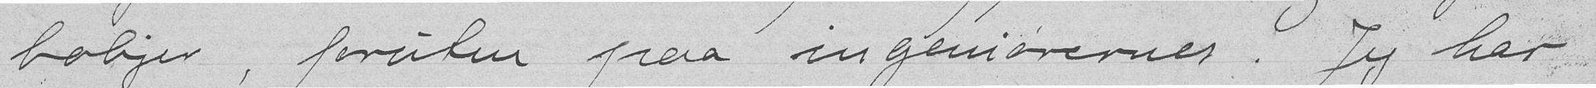boliger, foruten paa ingeniørernes. Jeg har
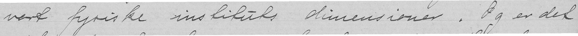vert fyriske instituts dimensioner. Og er det
